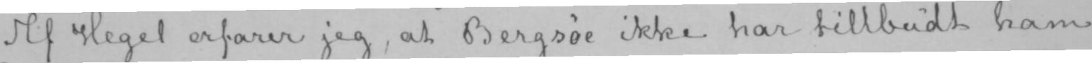Af Hegel erfarer jeg, at Bergsøe ikke har tillbudt ham
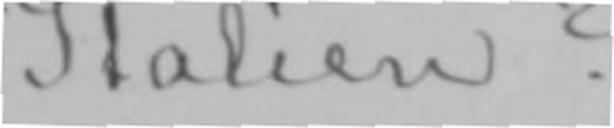Italien?
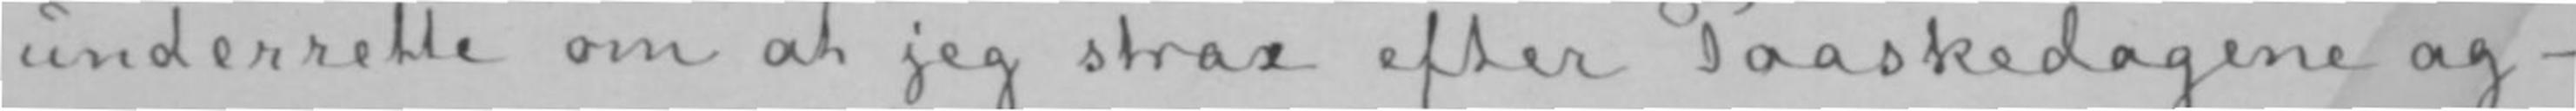underrette om at jeg strax efter Paaskedagen ag-
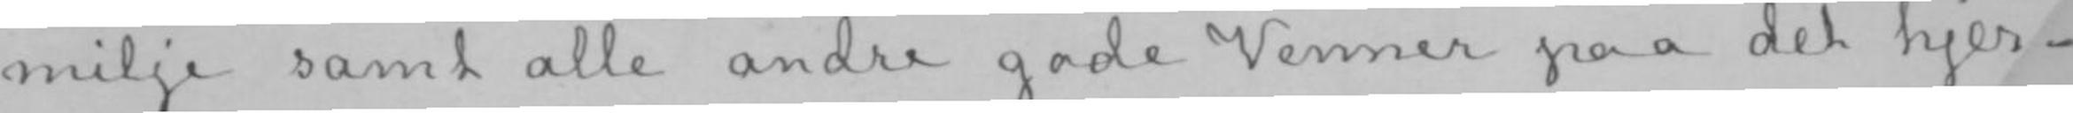milje samt alle andre gode Venner paa det hjer-
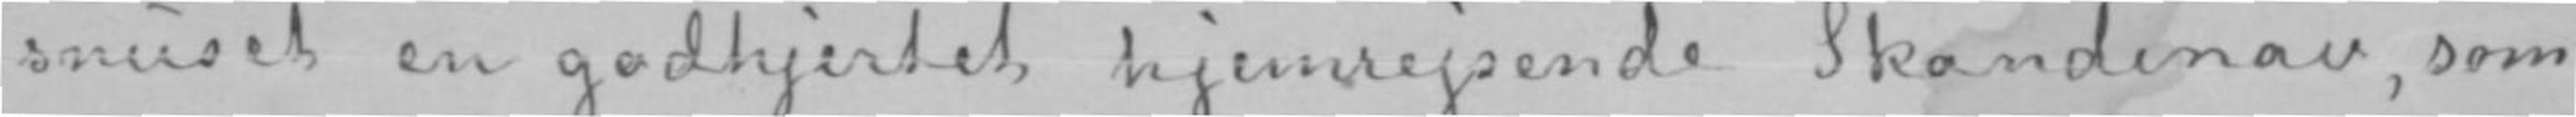snuset en godhjertet hjemrejsende Skandinav, som
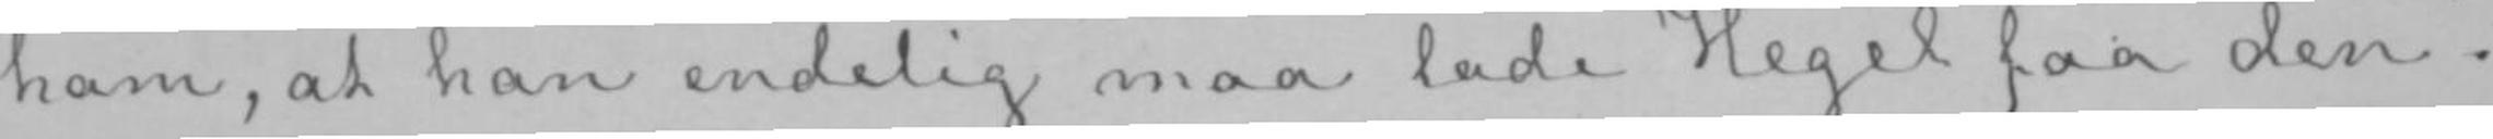ham, at han endelig maa lade Hegel faa den.
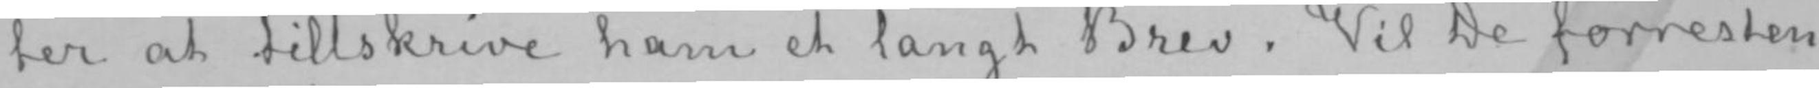ter at tillskrive ham et langt Brev. Vil De forresten
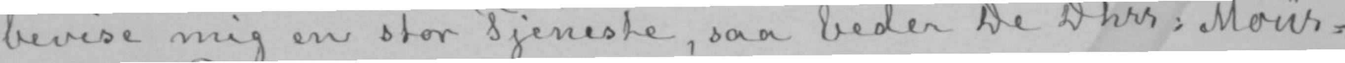bevise mig en stor Tjeneste, saa beder De Dhrr: Mour-
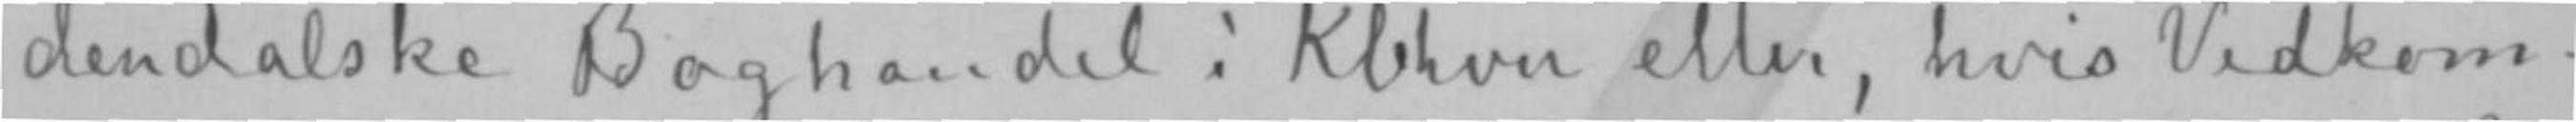dendalske Boghandel i Kbhvn eller, hvis Vedkom-
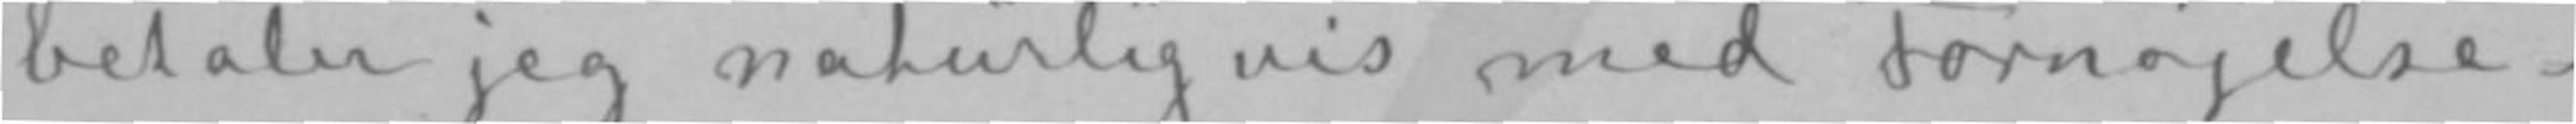betaler jeg naturligvis med Fornøjelse.
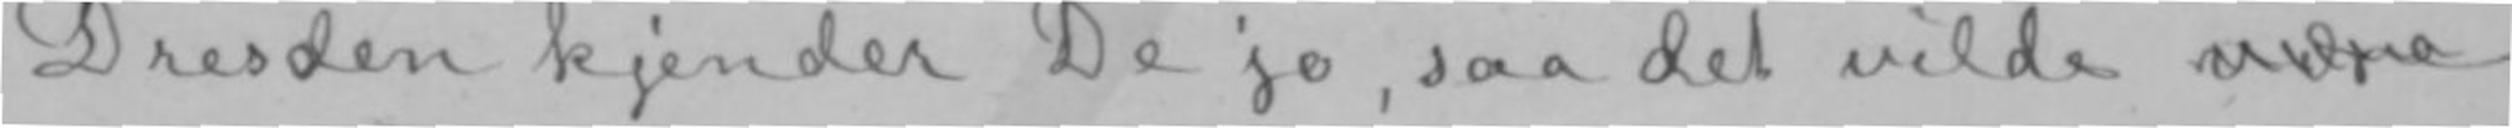Dresden kjender De jo, saa det vilde være
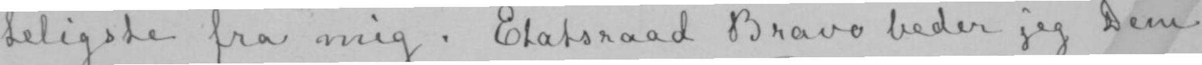teligste fra mig. Etatsraad Bravo beder jeg Dem
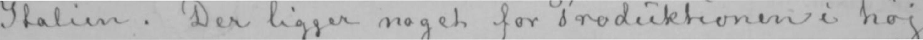Italien. Der ligger noget for Produktionen i høj
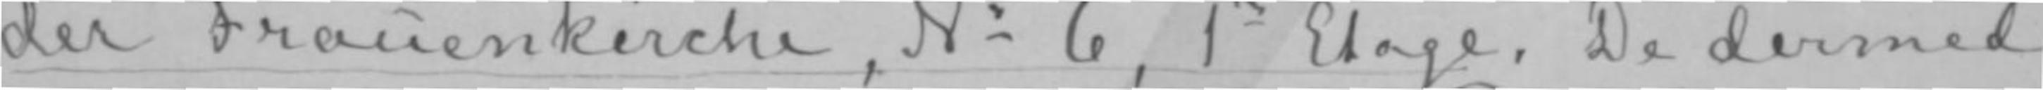der Frauenkirche, No 6, 1te Etage. De dermed
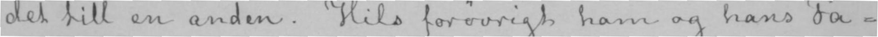det till en anden. Hils forøvrigt ham og hans Fa-
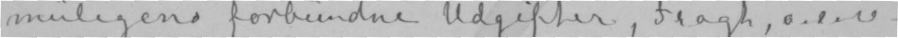muligens forbundne Udgifter, Fragt, o. s. v-
